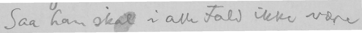Saa han skal i alle Fald ikke være
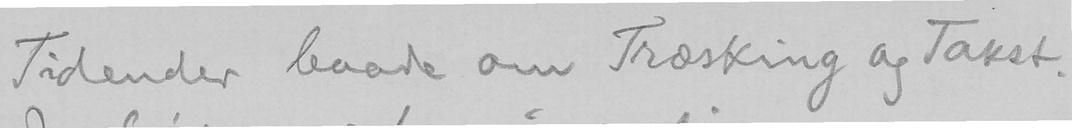Tidender baade om Træsking og Takst.
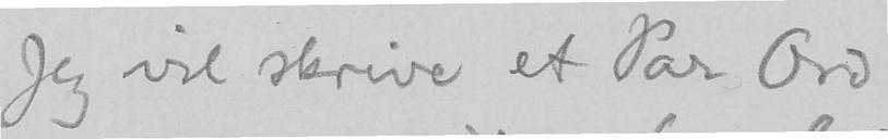Jeg vil skrive et Par Ord
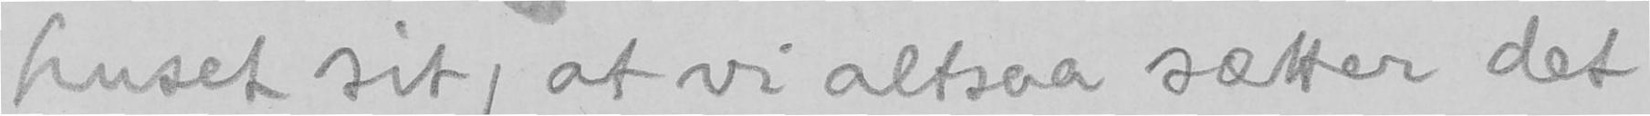huset sit, at vi altsaa sætter det
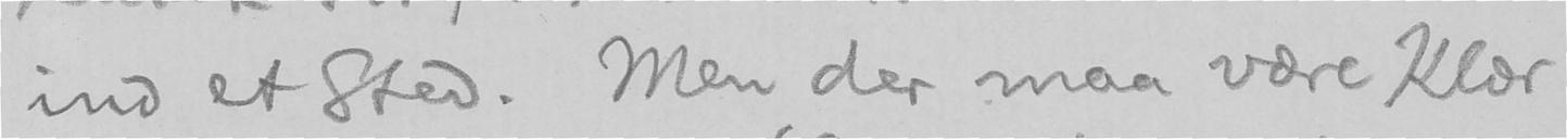ind et Sted. Men der maa være Klær
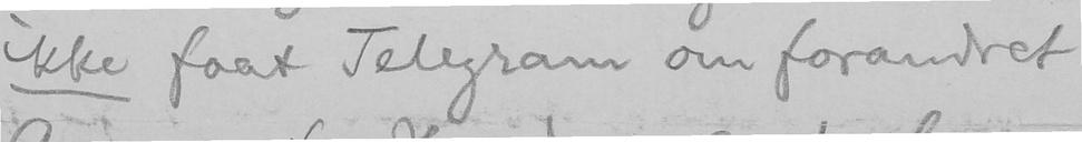ikke faat Telegram om forandret
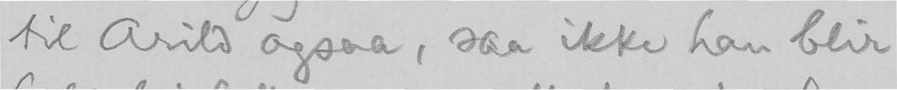til Arild ogsaa, saa ikke han blir
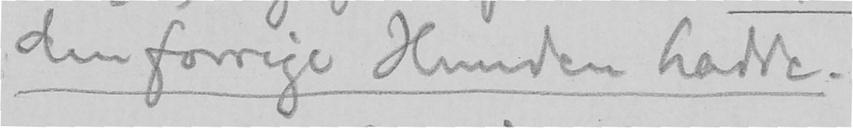den forrige Hunden hadde.
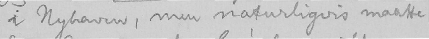i Nyhaven, men naturligvis maatte
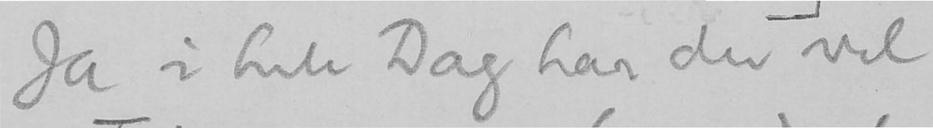Ja i hele Dag har du vel
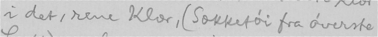i det, rene Klær, (Sækketøi fra øverste
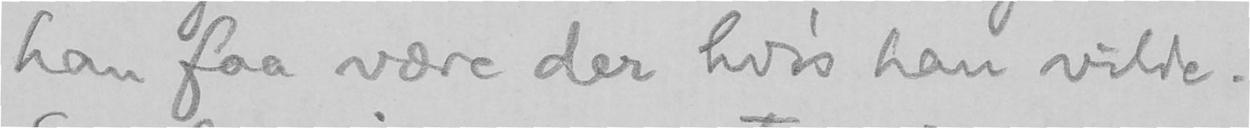han faa være der hvis han vilde.
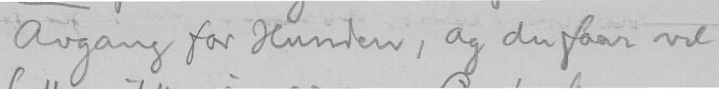Avgang for Hunden, og du faar vel
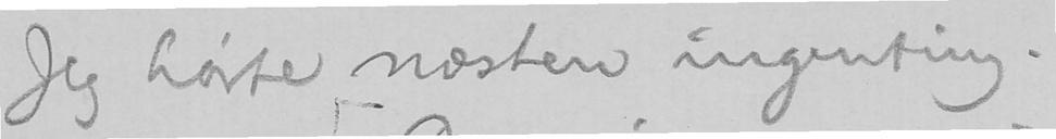Jeg hørte næsten ingenting.
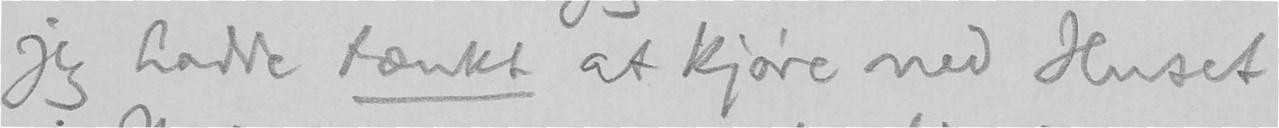jeg hadde tænkt at kjøre ned Huset
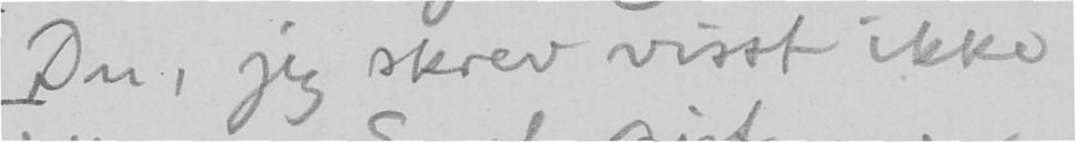Du, jeg skrev visst ikke
